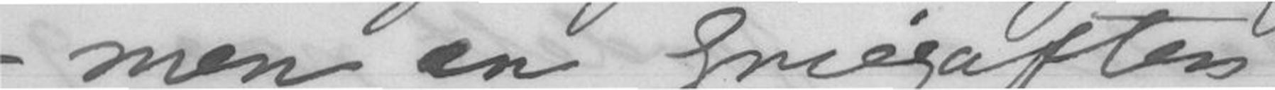- men en Griegaften
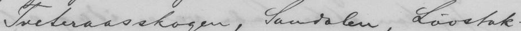Tveteraasskogen, Sandalen, Løvstak-
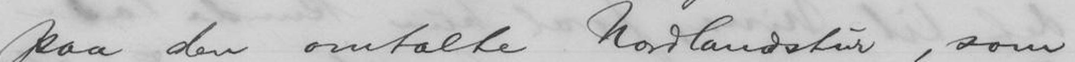paa den omtalte Nordlandstur, som
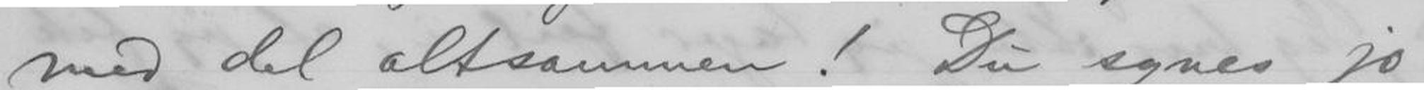med det altsammen! Du synes jo,
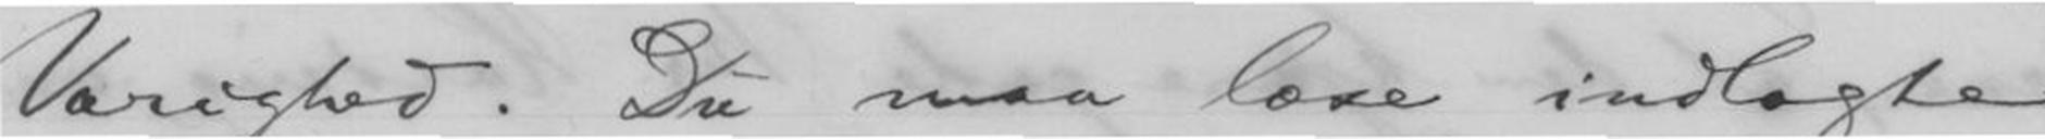Varighed. Du maa læse indlagte
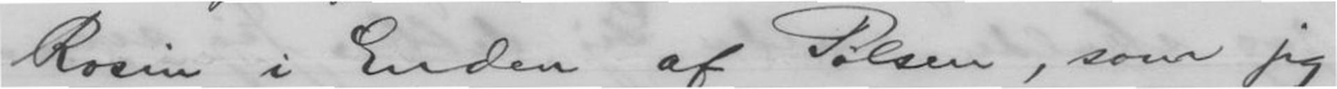Rosin i Enden af Pølsen, som jeg
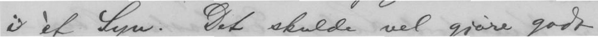i èt Syn. Det skulde vel gjøre godt
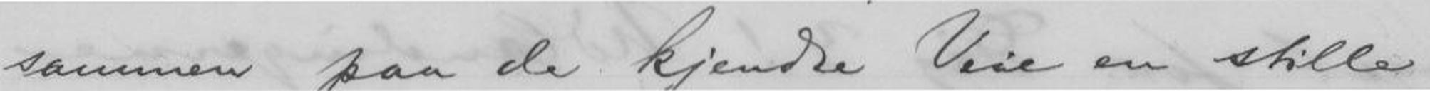sammen paa de kjendte Veie en stille
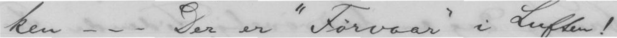ken - - - Der er "Førvaar" i Luften!
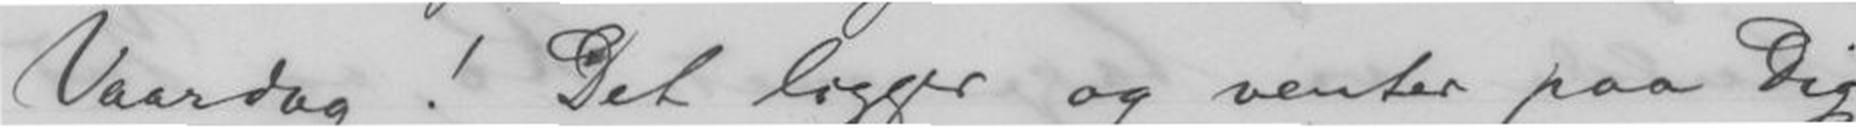Vaardag! Det ligger og venter paa Dig
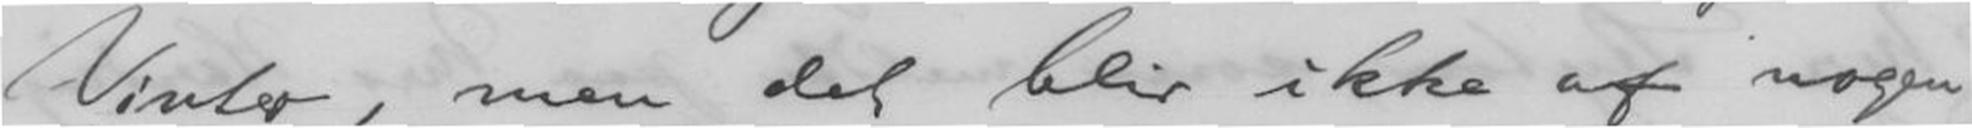Vinter, men det blir ikke af nogen
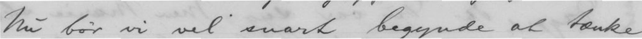Nu bør vi vel snart begynde at tænke
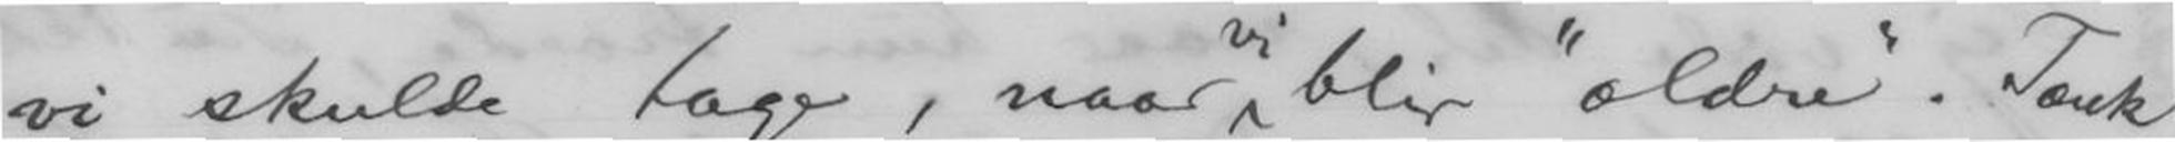vi skulde tage, naar vi blir "ældre". Tænk
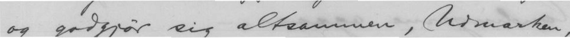og godgjør sig altsammen, Udmarken,
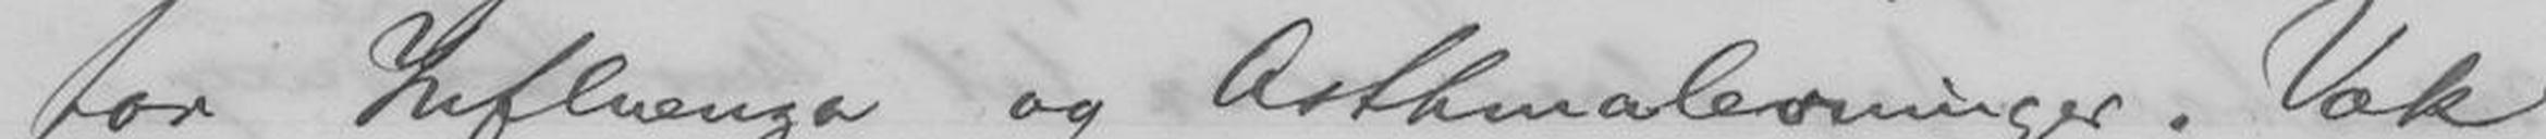for Influenza og Asthmalevninger. Væk
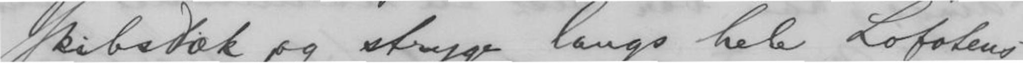skibsdæk og stryge langs hele Lofotens
